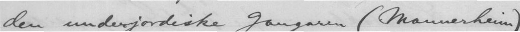den underjordiske Gangaren (Mannerheim)
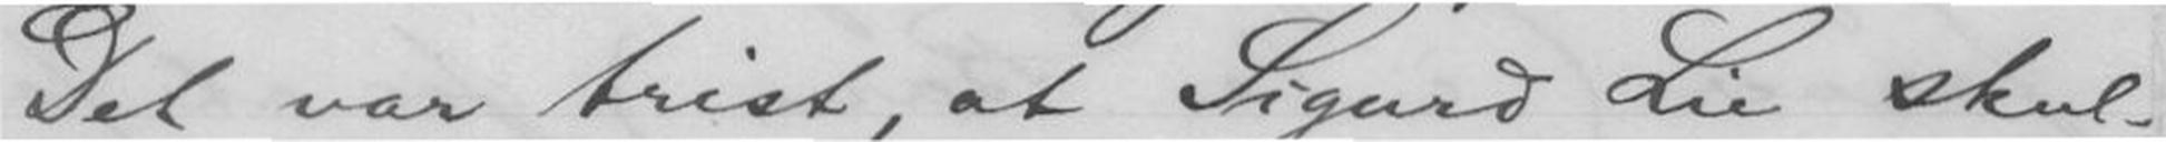Det var trist, at Sigurd Lie skul-
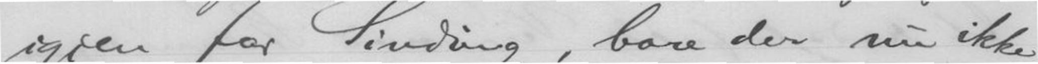igjen for Sinding, bare der nu ikke
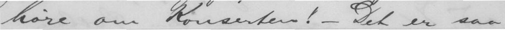høre om Konserten! - Det er saa
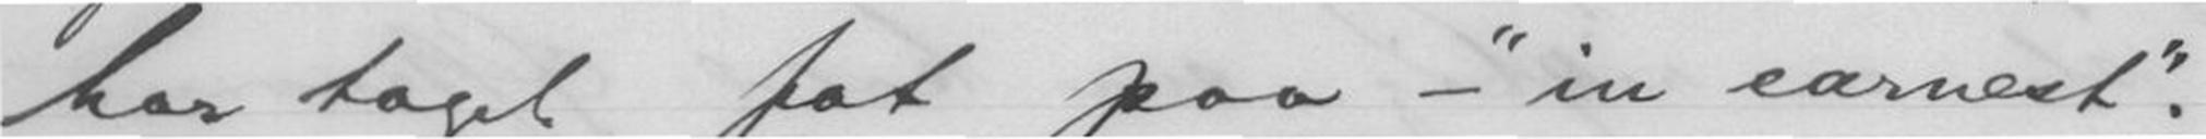har taget fat paa - "in earnest".
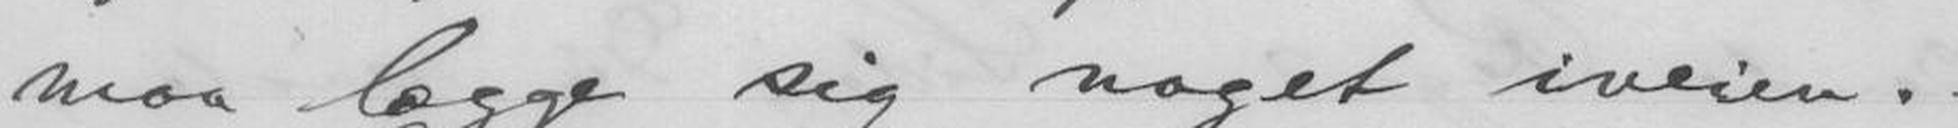maa lægge sig noget iveien. -
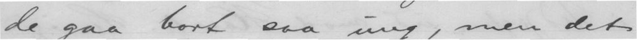de gaa bort saa ung, men det
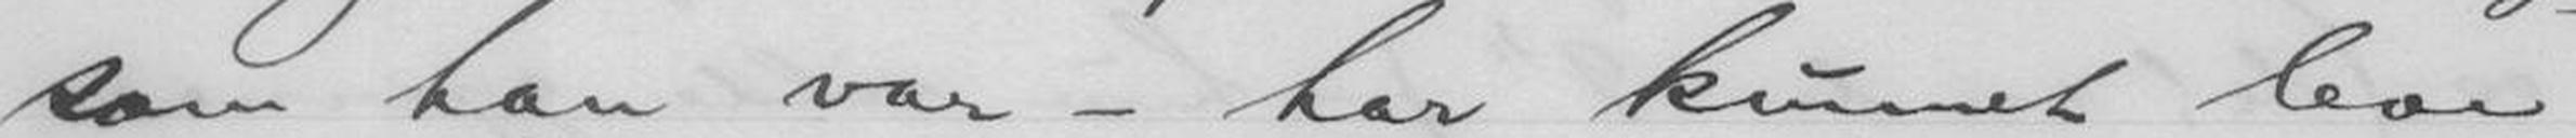som han var - har kunnet leve
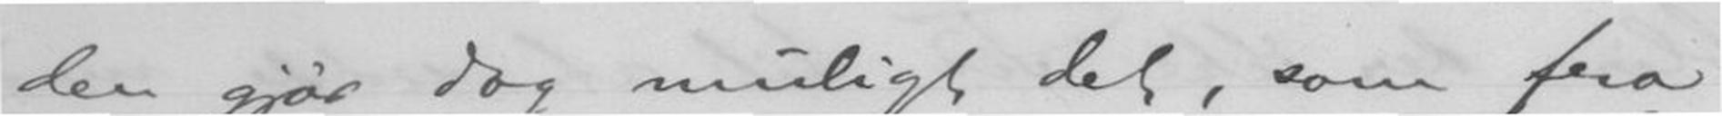den gjør dog muligt det, som fra
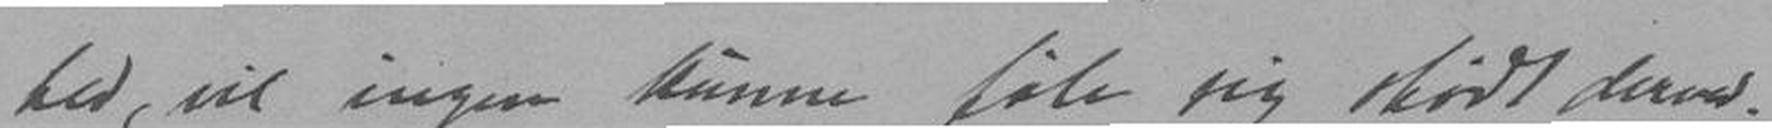hed, vil ingen kunne føle sig skødt derved.
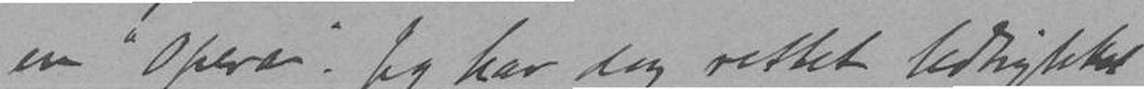en "Opera". Jeg har dog rettet Udtrykket
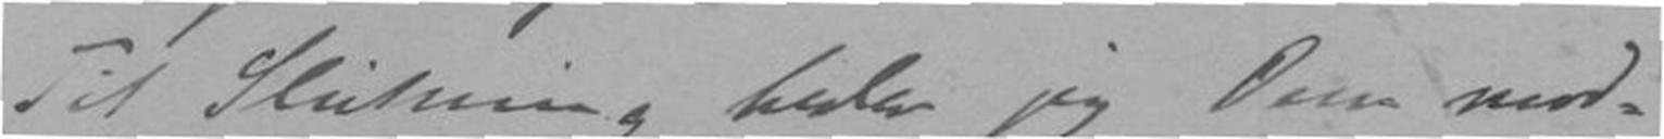Til Slutning beder jeg Dem mod-
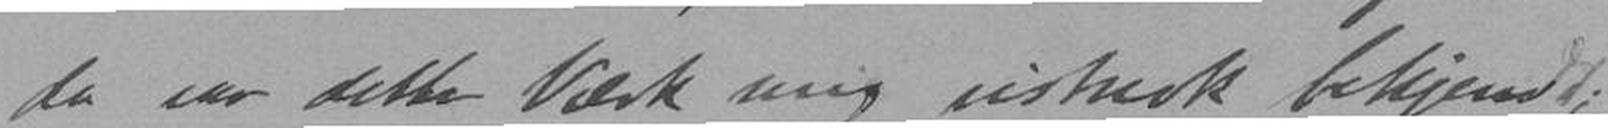da ser dette Værk mig vistnok bekjendt;
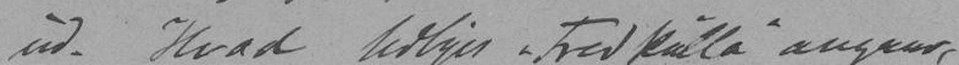ud. Hvad Udliges Fredkalla angaar,
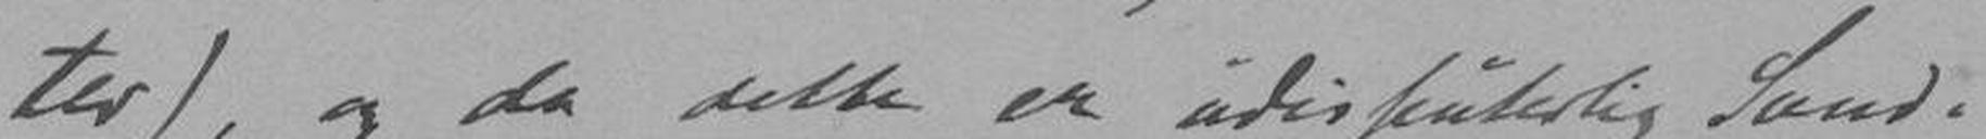ter), og da dette er udisputerlig Sand-
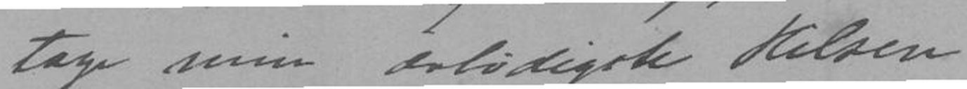tage min ærbødigste Hilsen
